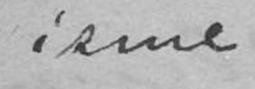isme
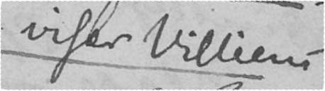viser Villiens
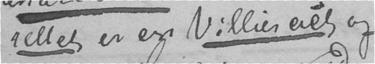ell el er en Villienes og
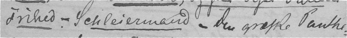Frihed – Schleiermand – Den græske Panthe-
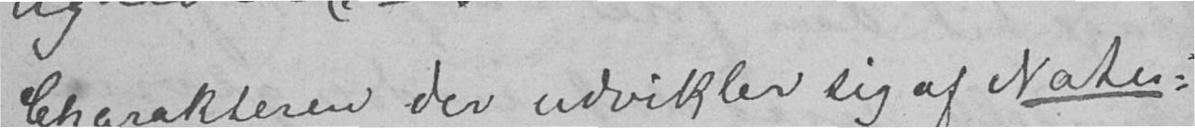Charakteren der udvikler sig af Natur-
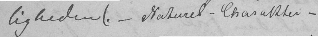ligheden (Naturel – Charakter –
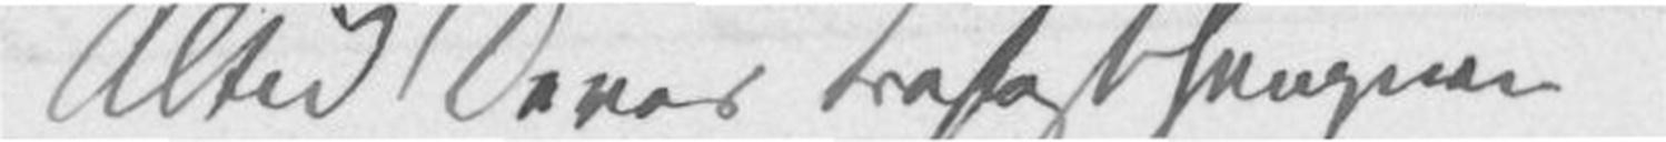Altid Deres trofast hengivne
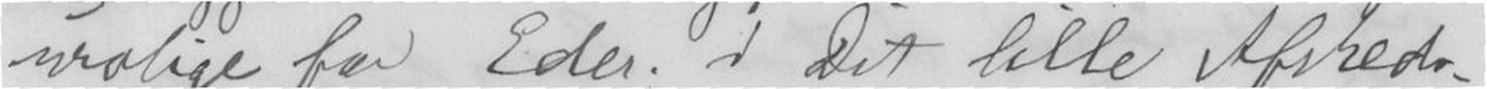urolige for Eder; i Dit lille Afskeds-
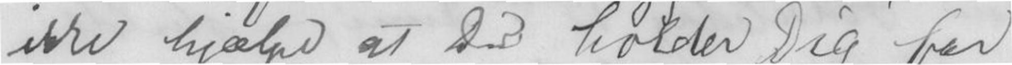ikke hjælpe at Du holder Dig for
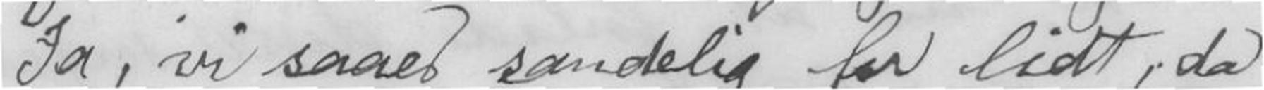- Ja, vi saaes sandelig for lidt, da
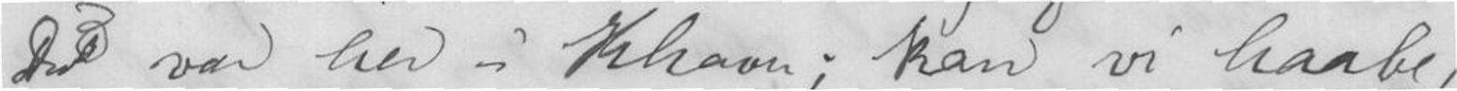Du var her i Khavn; kan vi haabe,
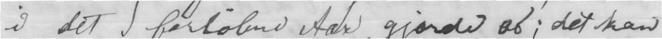i det forløbne Aar gjorde os; det kan
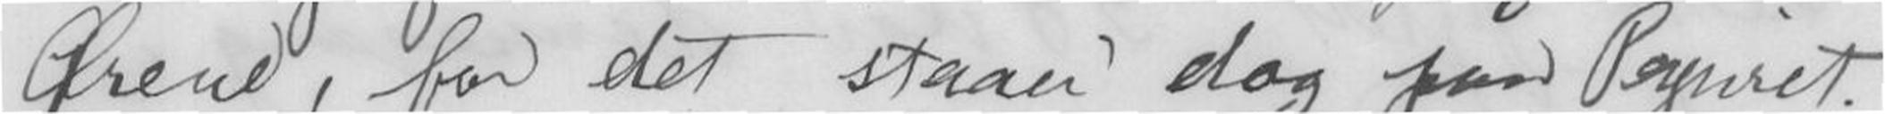Ørene, for det staaer dog paa Papiret.
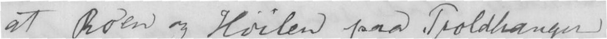at Roen og Hvilen paa Troldhaugen
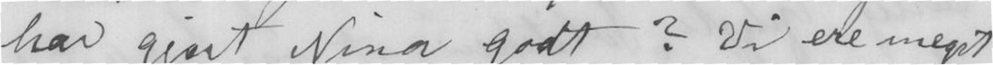har gjort Nina godt? Vi ere meget
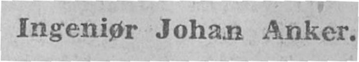Ingeniør Johan Anker.
